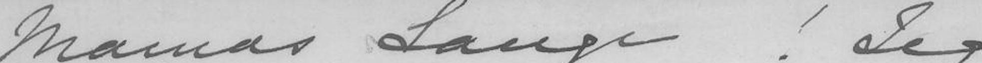Mamas Lauje! Jeg
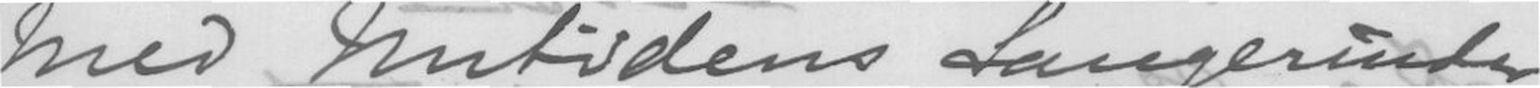med nutides Sangerinder,
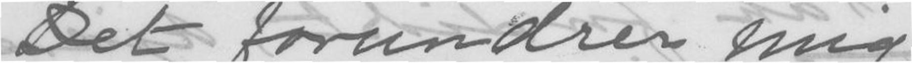Det forundre mig
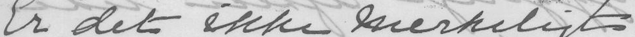Er det ikke merkeligt,
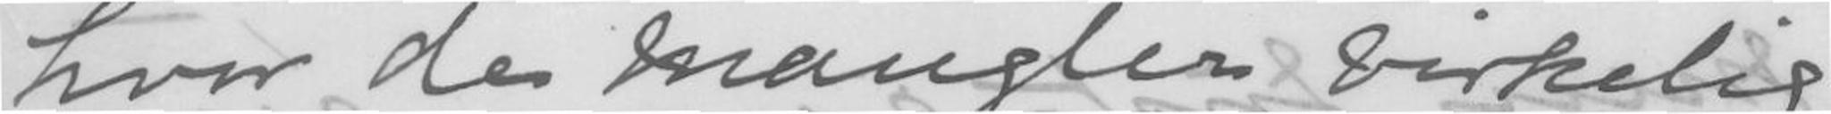hvor de mangler virkelig
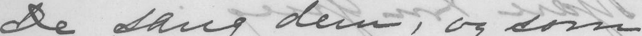De sang dem, og som
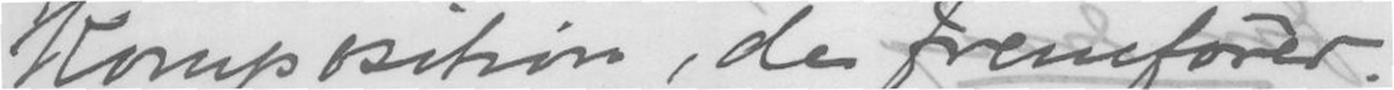Konposition, de fremfører.
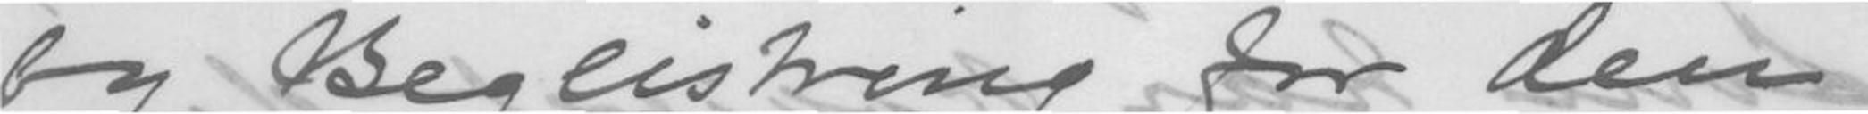og Begeistring for den
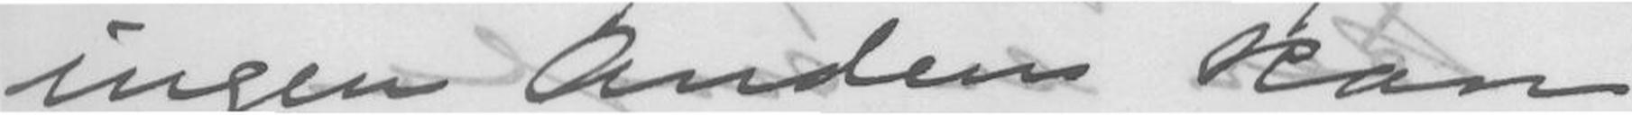ingen Anden kan
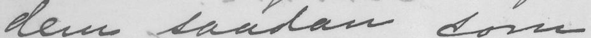dem, saadan som
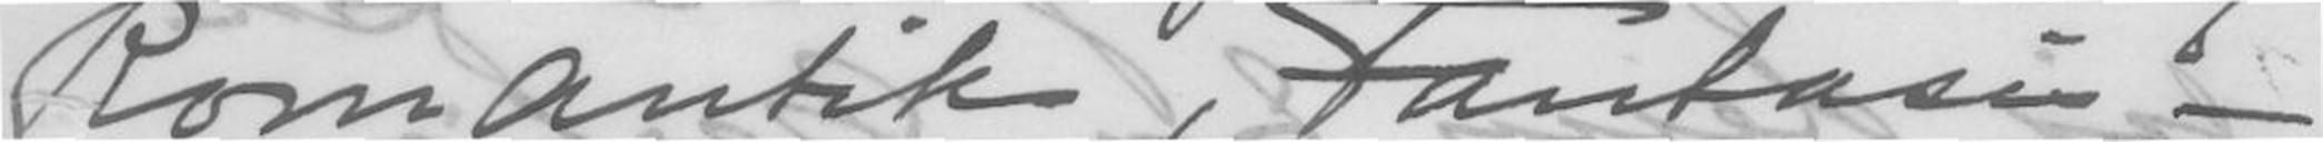Romantik, Fantasi -
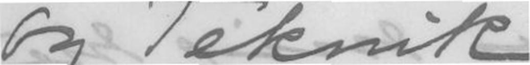og Teknik
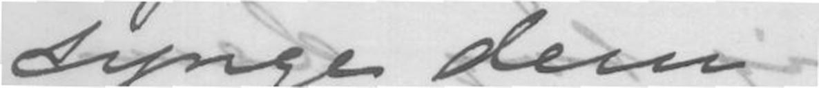synge dem
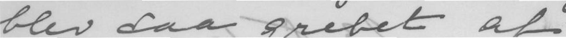blev saa grebet af
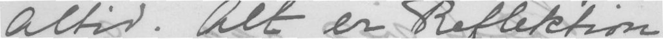altid. Det er Reflektion
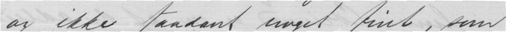og ikke saadant noget fint, som
